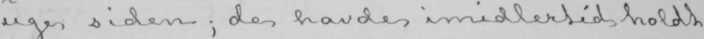uge siden; de havde imidlertid holdt
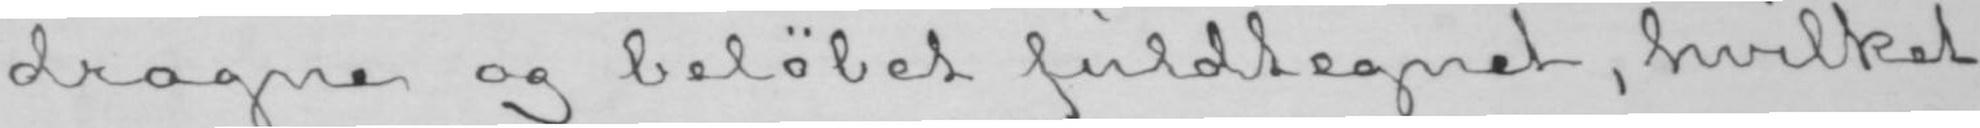dragne og beløbet fuldtegnet, hvilket
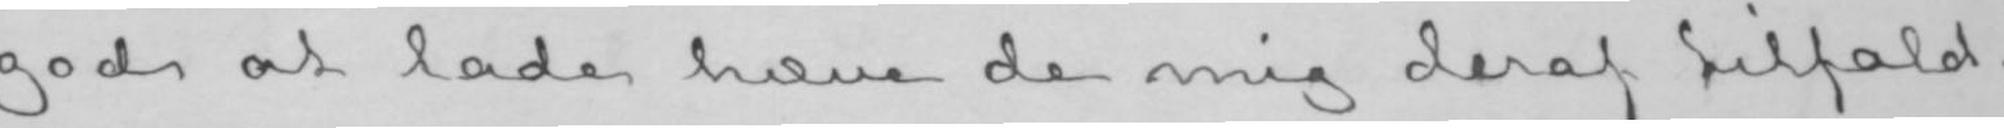god at lade hæve de mig deraf tilfald-
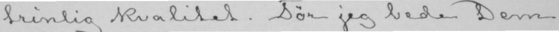trinlig kvalitet. Tør jeg bede Dem
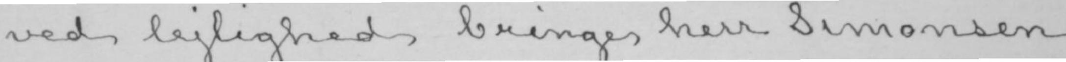ved lejlighed bringe herr Simonsen
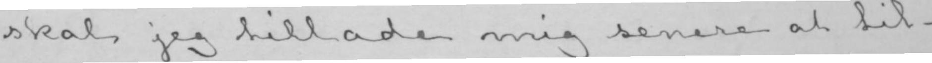skal jeg tillade mig senere at til-
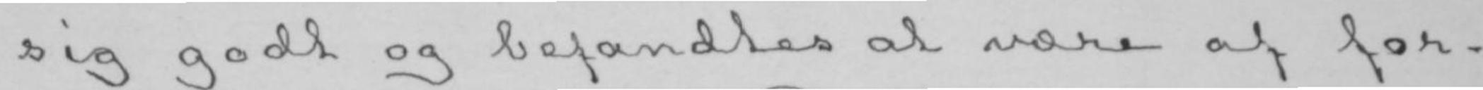sig godt og befandtes at være af for-
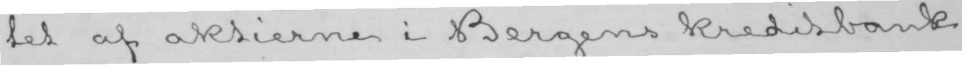tet af aktierne i Bergens kreditbank,
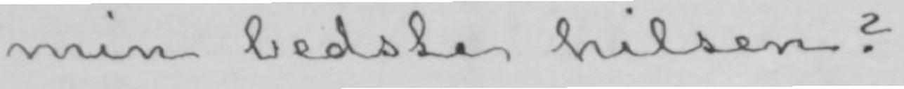min bedste hilsen?
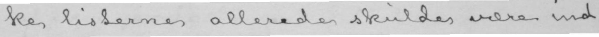ke listerne allerede skulde være ind-
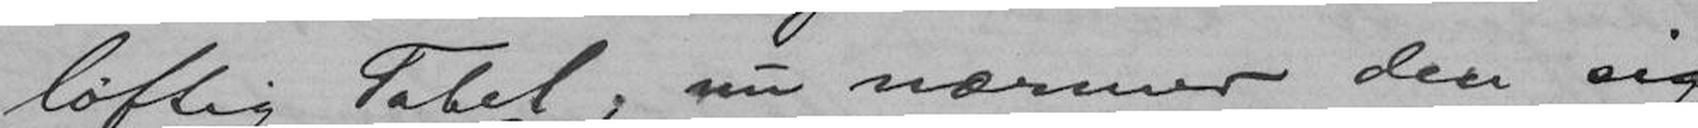løftig Tabel, nu nærmer den sig
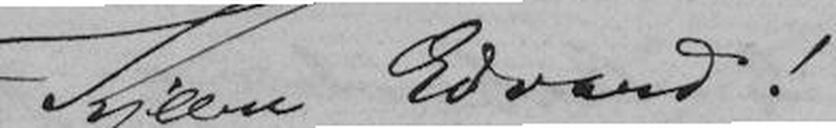Kjære Edvard!
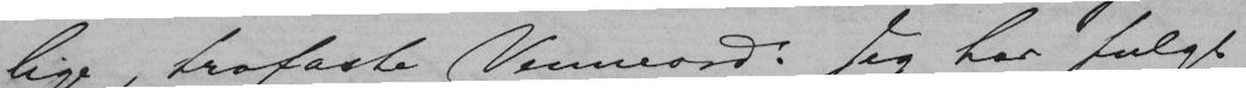lige, trofaste Venneord. Jeg har fulgt
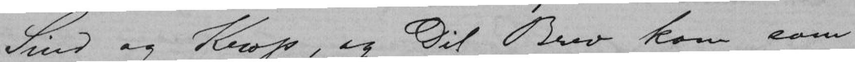Sind og Krop, og Dit Brev kom som
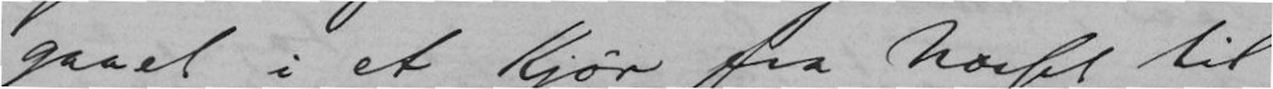gaaet i et Kjør fra Næsset til
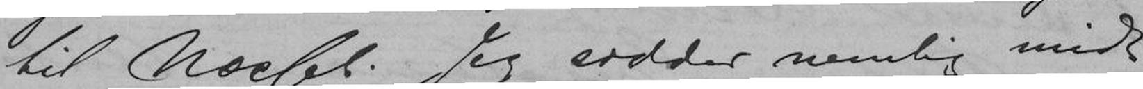til Næsset. Jeg sidder nemlig midt
